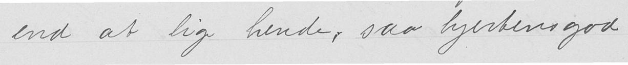end at lige hende, saa hjertens god
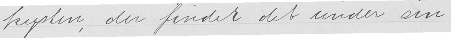kysten, der finder det under sin
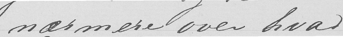nærmere over hvad
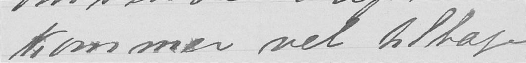kommer vel tilbage
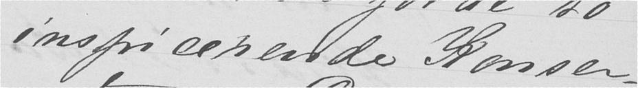inspicerende Konser-
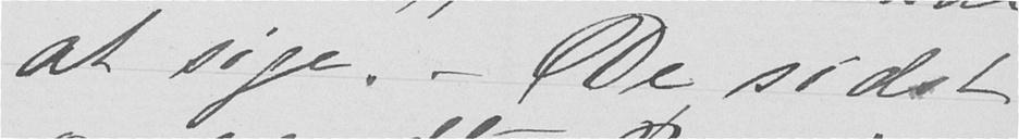at sige. – De sidst
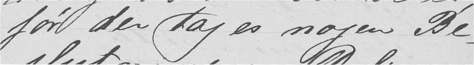før der tages nogen Be-
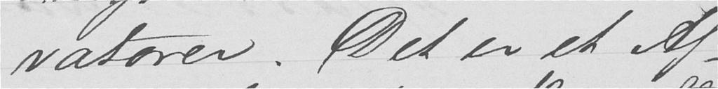vatorer. Det er et Af-
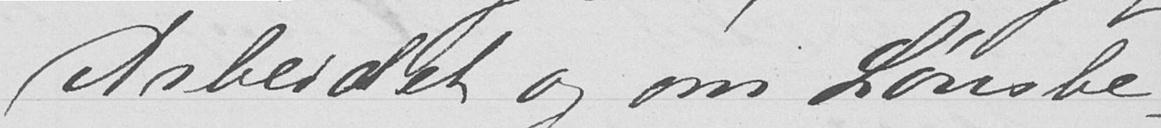Arbeidet og om Lønsbe-
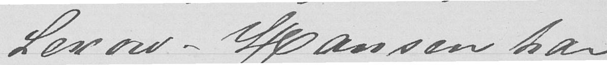Lexow-Hansen har
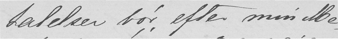talelser bør efter min Me-
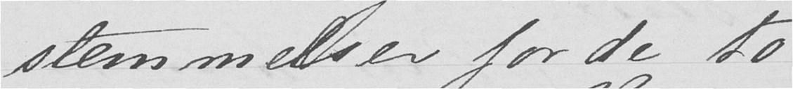stemmelser for de to
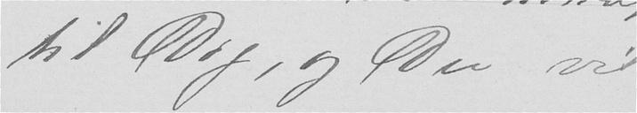til Dig, og Du vil
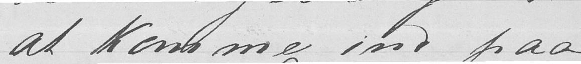at komme ind paa
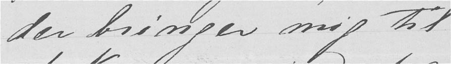der bringer mig til
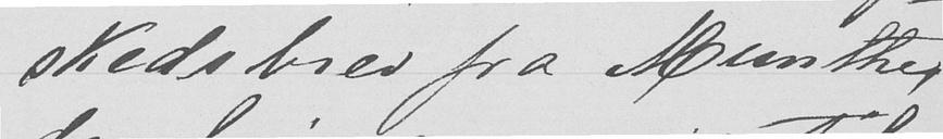skedsbrev fra Munthe,
